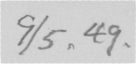9/5. 49.
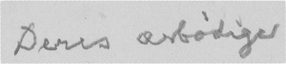Deres ærbødige
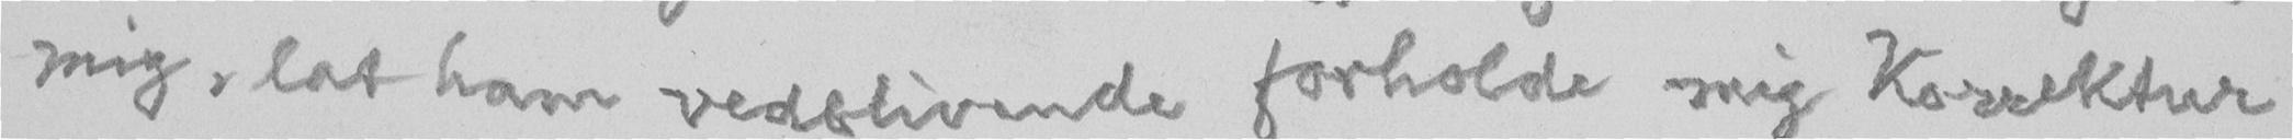mig, lat ham vedblivende forholde mig Korrektur
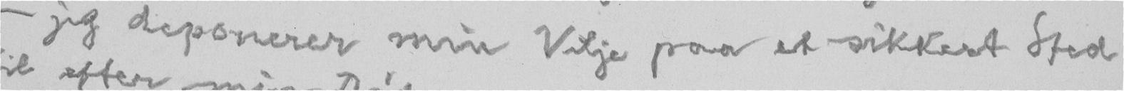- jeg deponerer min Vilje paa et sikkert Sted
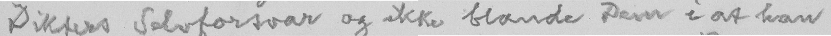Dikters Selvforsvar og ikke blande Dem i at han
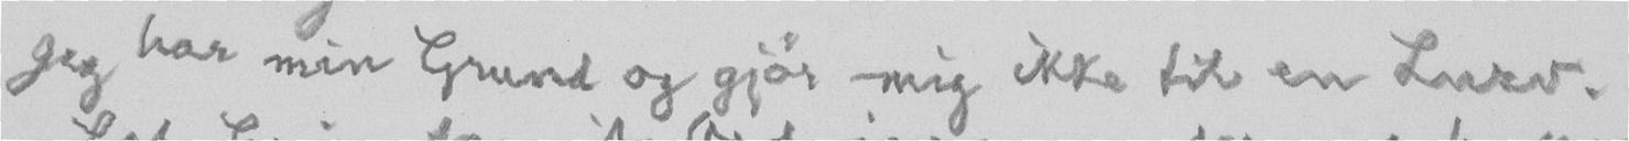Jeg har min Grund og gjør mig ikke til en Lurv.
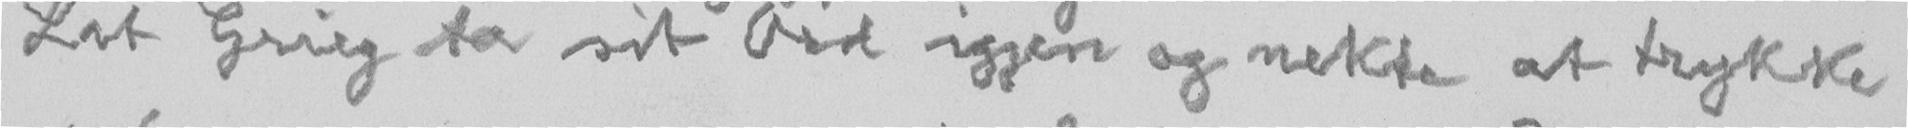Lat Grieg ta sit Ord igjen og nekte at trykke
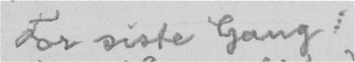For siste Gang:
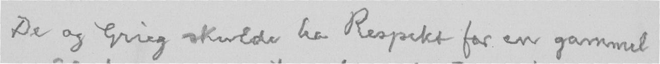De og Grieg skulde ha Respekt for en gammel
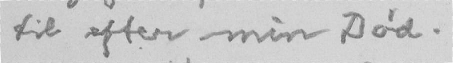til efter min Død.
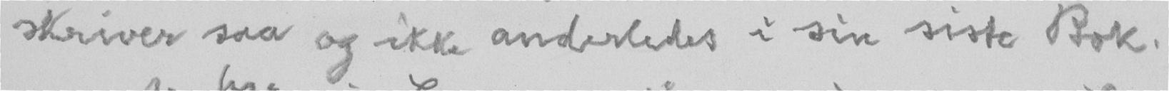skriver saa og ikke anderledes i sin siste Bok.
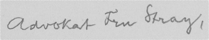Advokat Fru Stray,
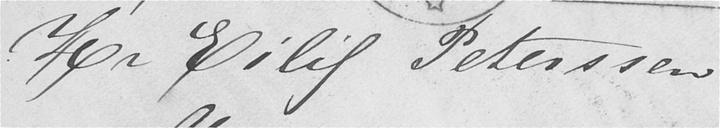Hr Eilif Peterssen
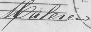Maleren
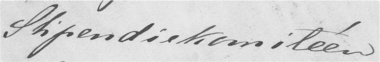Stipendiekomitéen
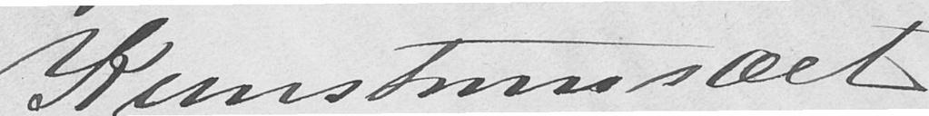Kunstmusæet
